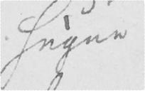hugne
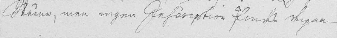Steene, men ingen Inscription findes derpaa -
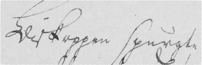Biskoppen spurgte
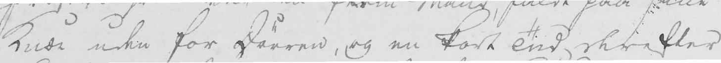Knær uden for Dørren, og en kort Tiid derefter
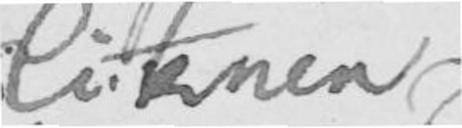liknen
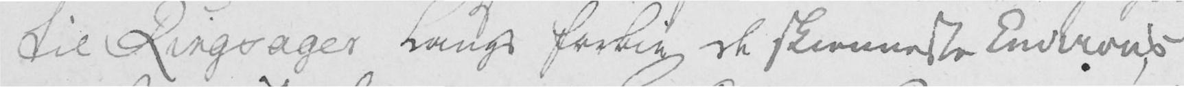til Ringsager langs forbie de skiønneste Endvious
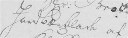Jordbærblade af
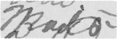Mads
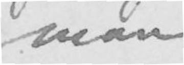man
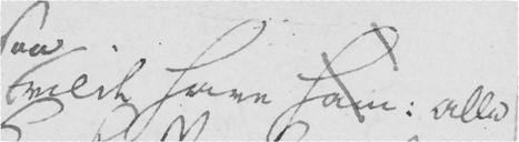vilde have ham: alle
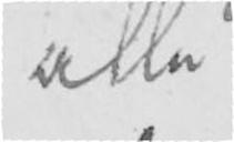alle
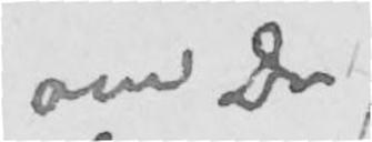om de
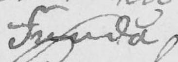Enda
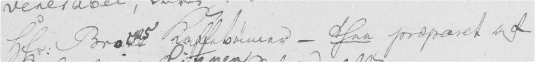Hr Brochs Kaffebønner - Een præparat af
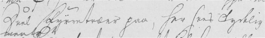Deel Fyrretræer paa, her sees tydelig
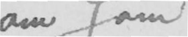om ham
